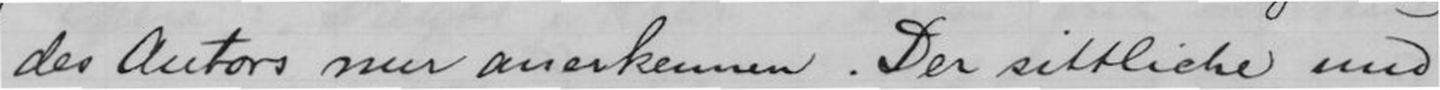des Autors nur anerkennen. Der sittliche und
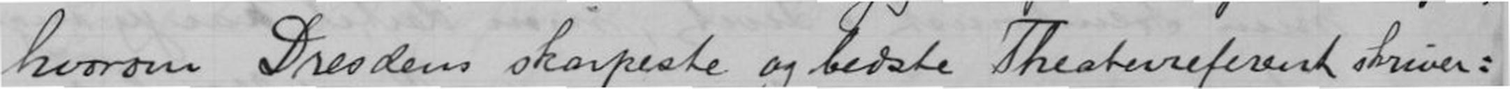hvorom Dresden skarpeste og bedste Theaterreferent skriver:
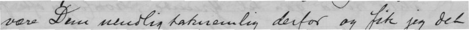være Dem uendelig taknemlig derfor og fik jeg det
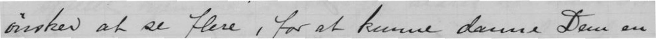ønsked at se flere, for at kunne danne Dem en
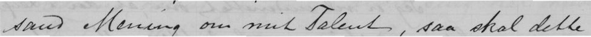sand Menig om mit Talent, saa skal dette
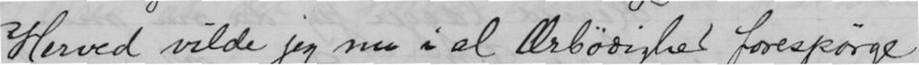Herved vilde jeg i al Ærbødighed forespørge
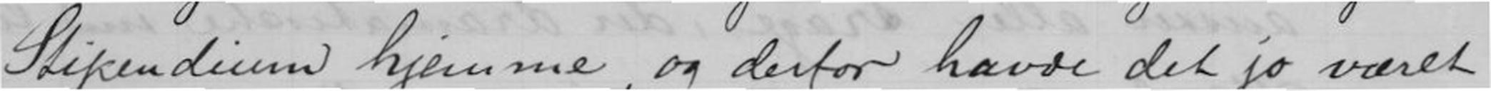Stipendium hjemme, og derfor havde det jo været
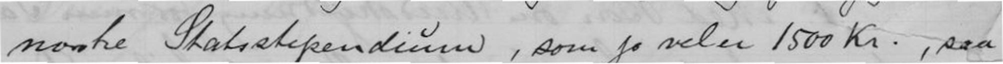norske Statsstipendium, som jo vel er 1500 Kr., saa
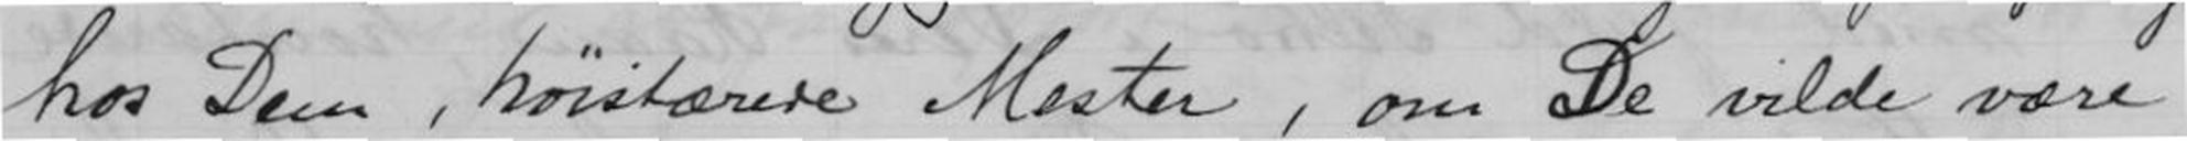hos Dem, høistærede Mester, om De vilde være
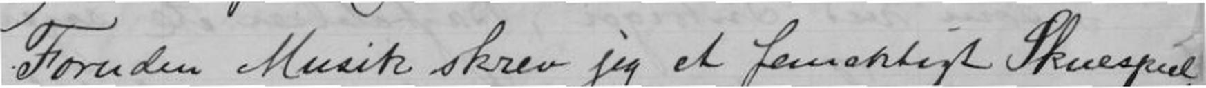Foruden Musik skrev jeg et femaktigt Skuespill
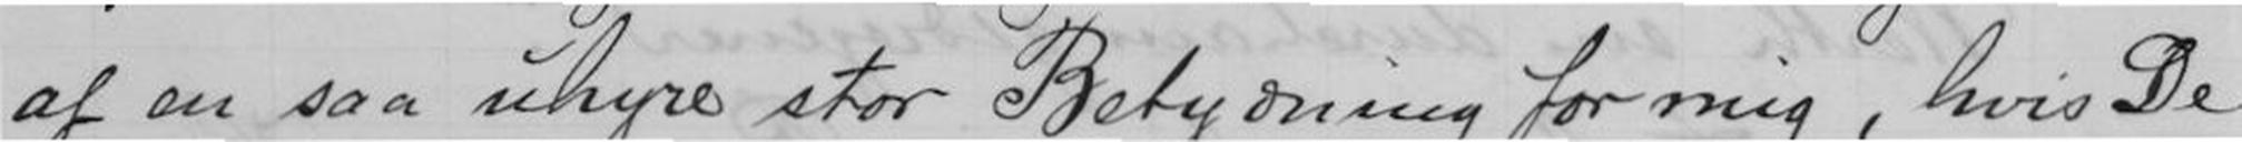af en saa uhyre stor Betydning for mig, hvis De
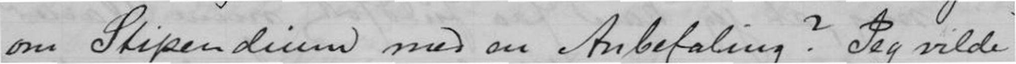om Stipendium med en Anbefaling? Jeg vilde
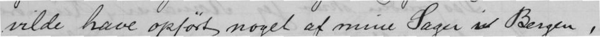vilde have opført noget af mine Sager i Bergen,
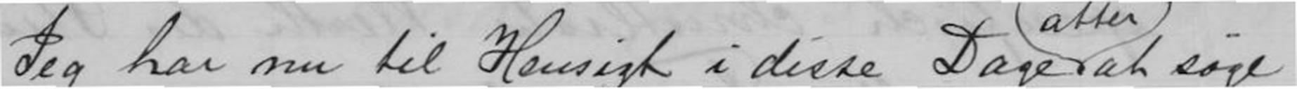Jeg har nu til Hensigt i disse Dage at søge
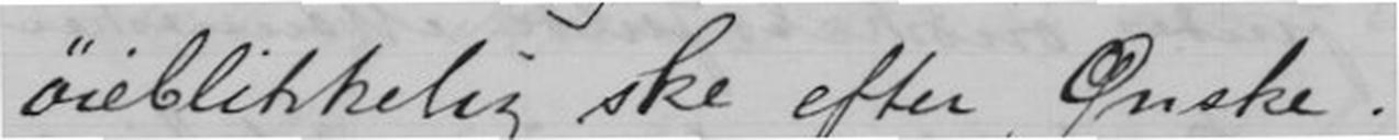øieblikkelig ske efter Ønske. -
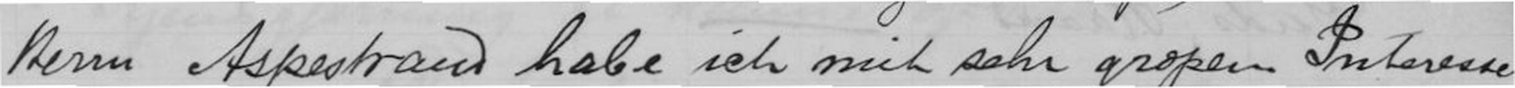Herrn Asphestrand habe hich mit sehr grossen Interesse
